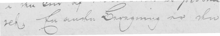ret; En anden beregning er den
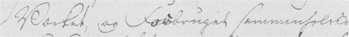Værket, og Forbruget sammenholdes
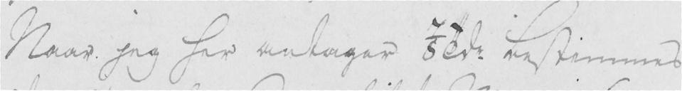Naar jeg her antager 7/8 Tdr bestemmes
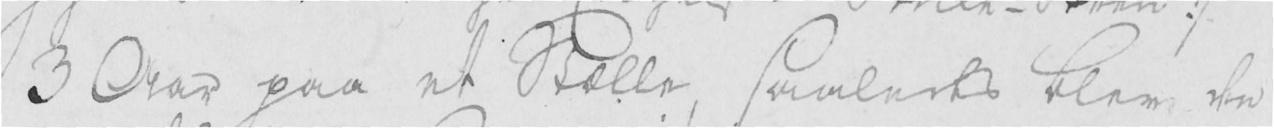3 Aar paa et Stælle, saaledes blev de
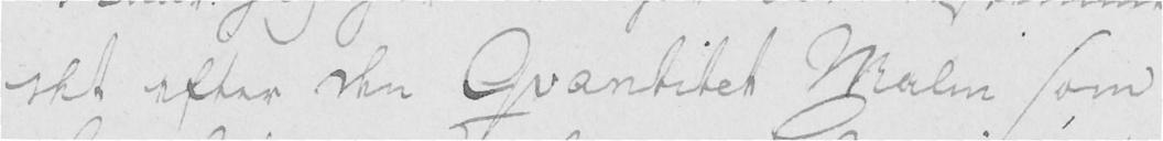det efter den Qvantitet Malm som
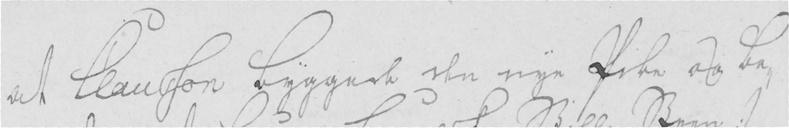at Clauson byggede den nye Pibe og be-
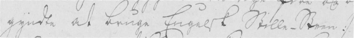gyndte at bruge Engelsk Stille-Steen :/
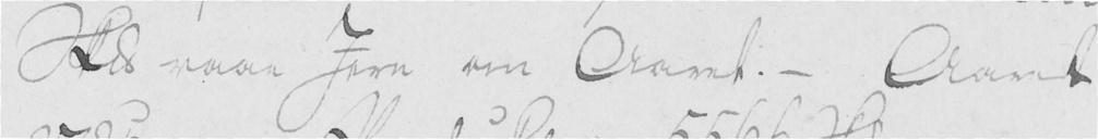Skpd raae Jern om Aaret. - Aaret
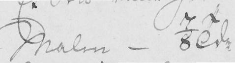Malm - 7/8 Tdr.
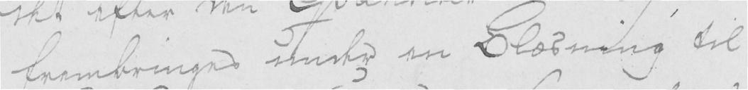frembringes under en blæsning til
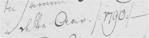i dette Aar. /: 1790 :/ -
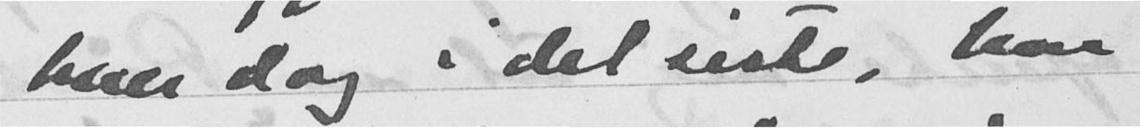hver dag i det siste, har
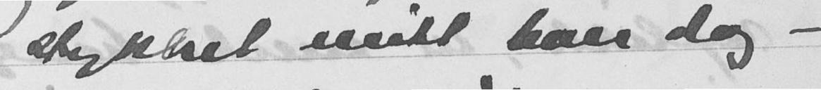stykket mitt hver dag -
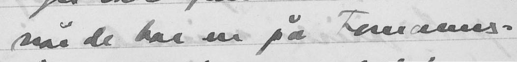når de har en på tomanns-
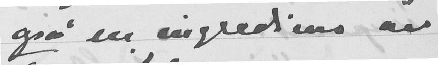også en ingrediens av
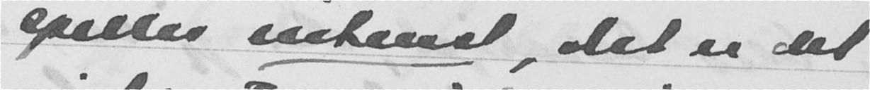spiller intenst, det er det
